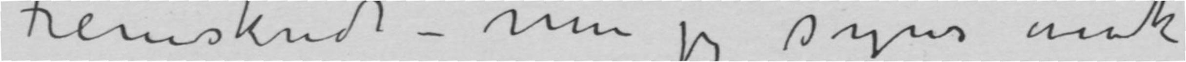Fremskridt – Men jeg syns nok
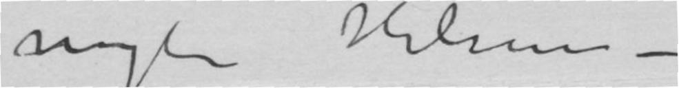nogle Hilsener –
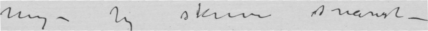mig – Jeg skriver snarest –
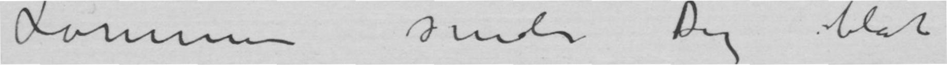Lommen sender Dig blot
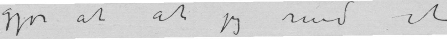gjør at at jeg med et
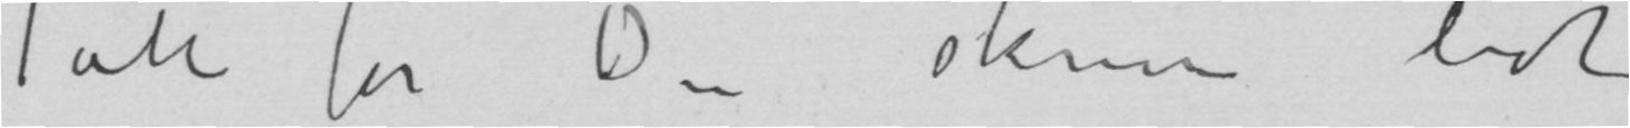Tak for Du skriver lidt
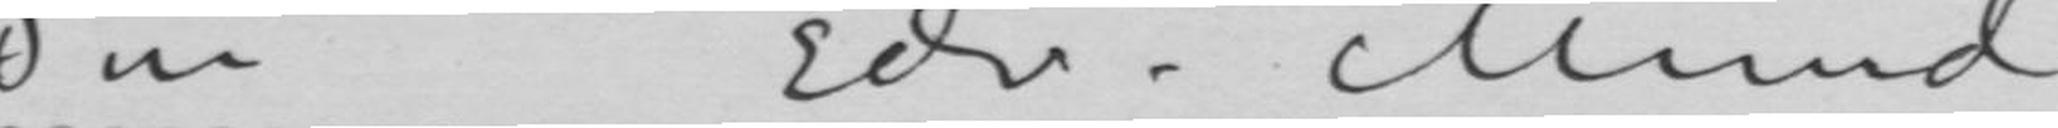Din Edv. Munch
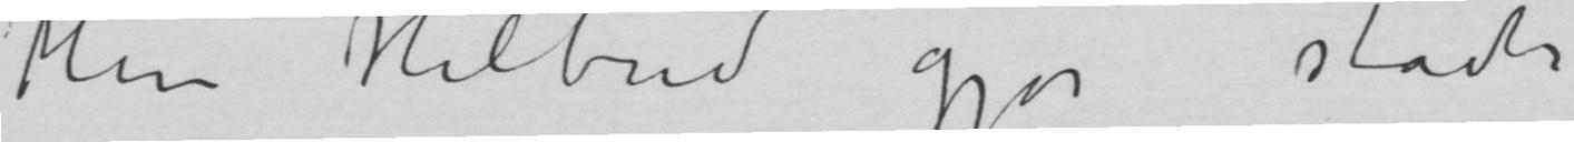Min Helbred gjør stadi
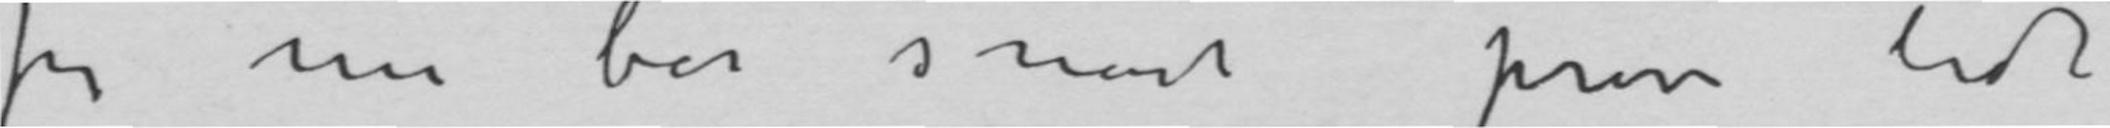jeg nu bør snart prøve lidt
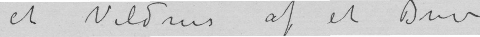et Vildnis af et Brev
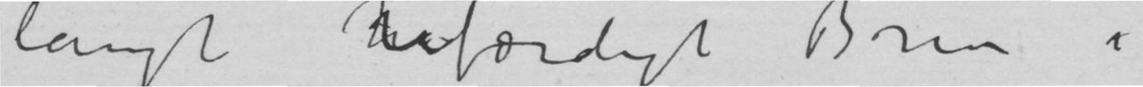langt hufærdigt Brev i
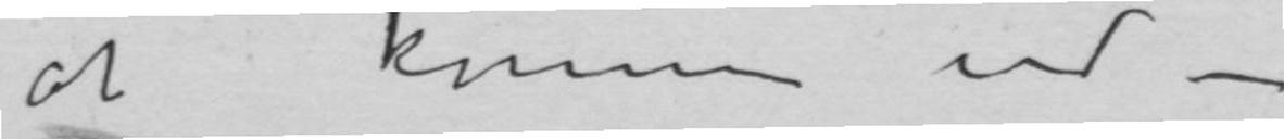at komme ud –
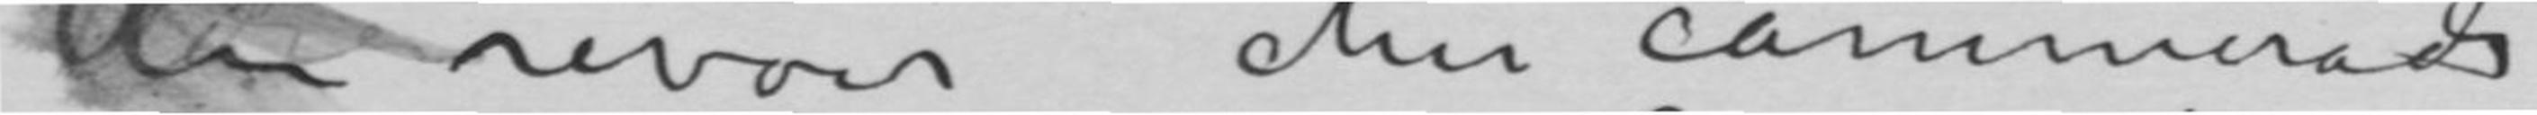Au revoir cher Cammerad
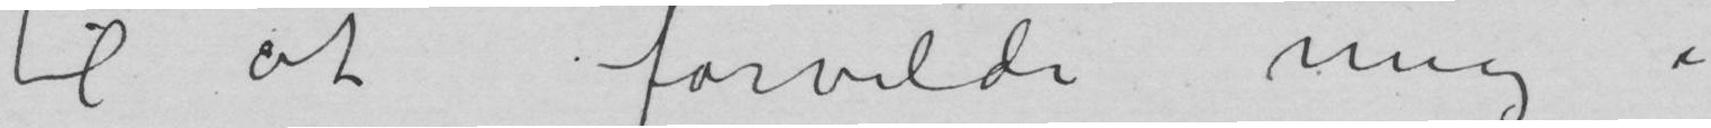til at forvilde mig i
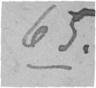65.
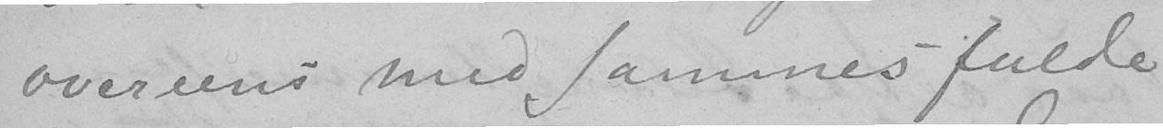overeens med sammes fulde
